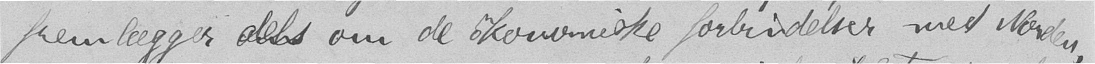fremlægger dels om de økonomiske forbindelser med Norden,
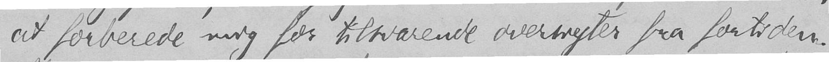at forberede mig for tilsvarende oversigter fra fortiden.
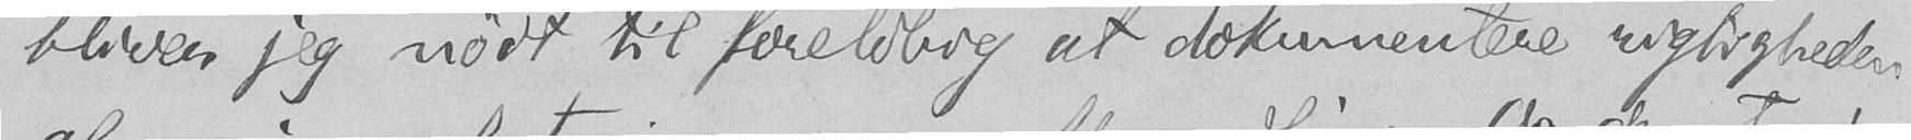bliver jeg nødt til foreløbig at dokumentere rigtigheden
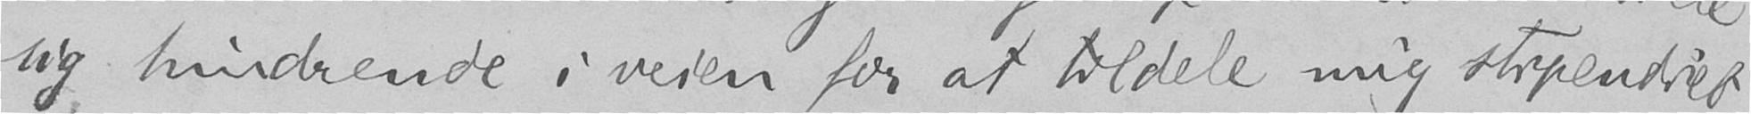sig hindrende i veien for at tildele mig stipendiet
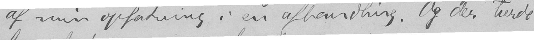af min opfatning i en afhandling. Og der turde
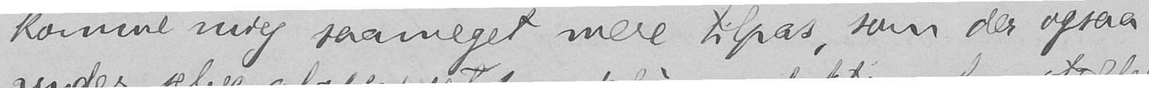komme mig saameget mere tilpas, som der ogsaa
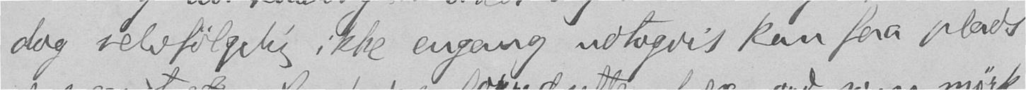dog selvfølgelig ikke engang udtagvis kan faa plads
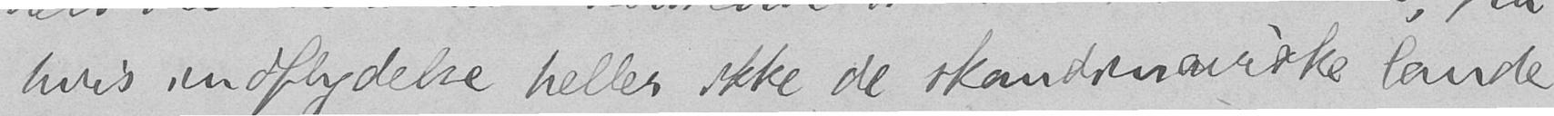hvis indflydelse heller ikke de skandinaviske lande
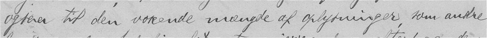ogsaa til den voxende mængde af oplysninger, som andre
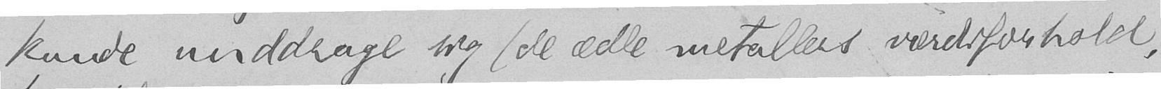kunde unddrage sig (de ædle metallers værdiforhold,
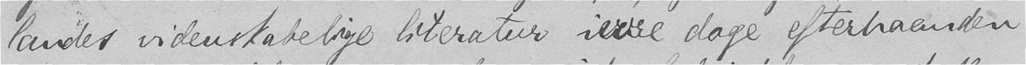landes videnskabelige literatur i vore dage efterhaanden
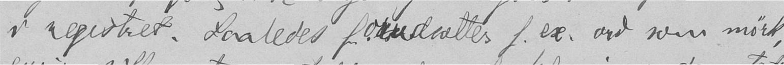i registret. Saaledes forudsættes f.ex. ord som mørk,
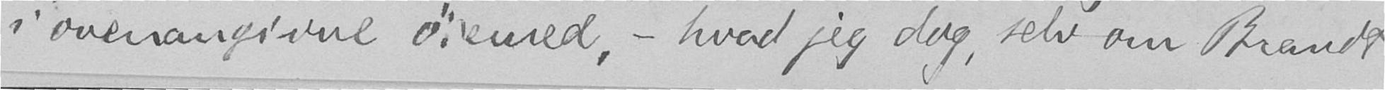i ovenangivne øiemed, - hvad jeg dog, selv om Brandt
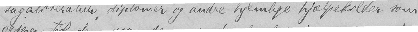sagaliteratur, diplomer og andre hjemlige hjælpekilder som
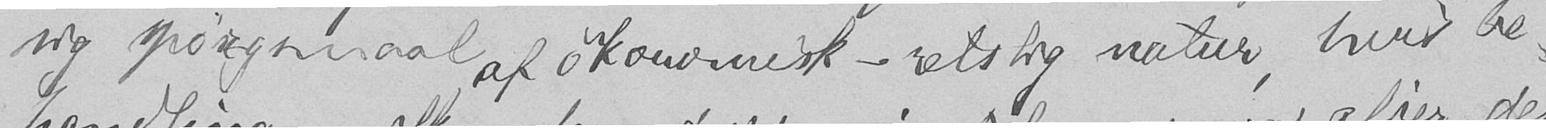sig spørgsmaal af økonomisk-retslig natur, hvad be-
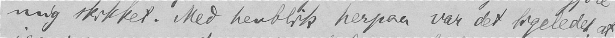mig skikket. Med henblik herpaa var det ligeledes, at
